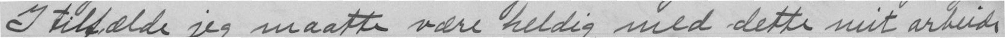I tilfælde jeg maatte være heldig med dette mit arbeide,
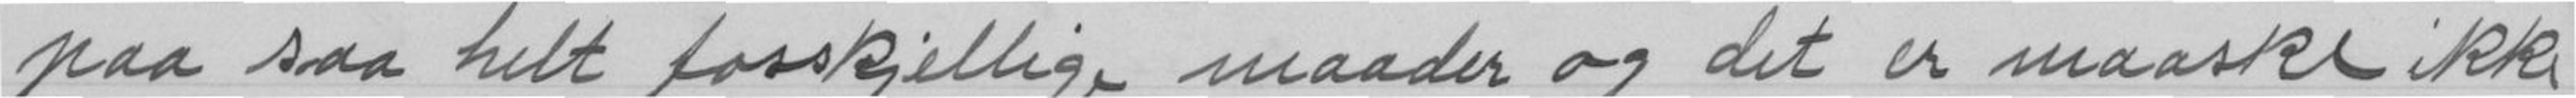paa saa helt forskjellig maader og det er maaske ikke
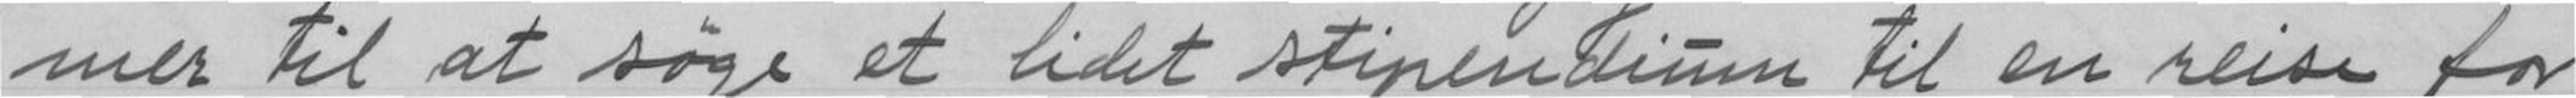mer til at søge et lidet stipendium til en reise for
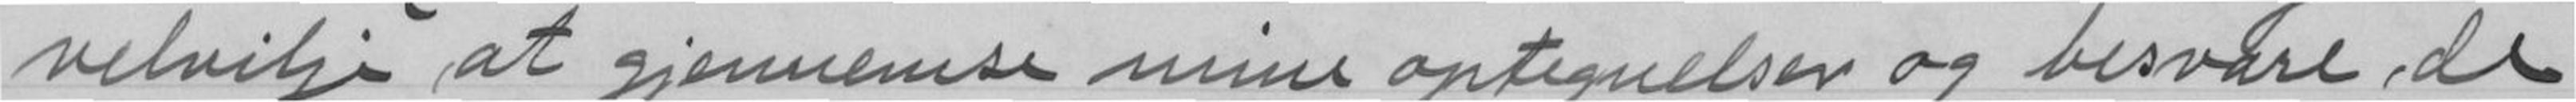velvilje at gjennemse mine optegnelser og besvare de
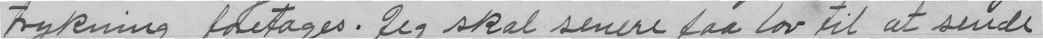trykning foretages. Jeg skal senere faa lov til at sende
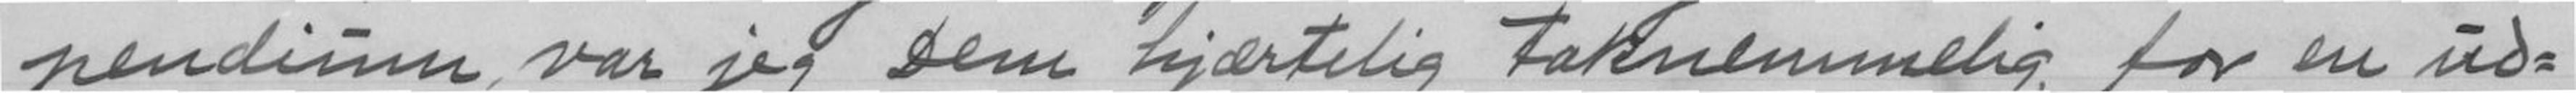pendium var jeg Dem hjærtelig taknemmelig for en ud-
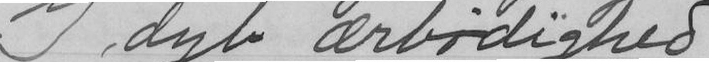I dyb ærbødighed
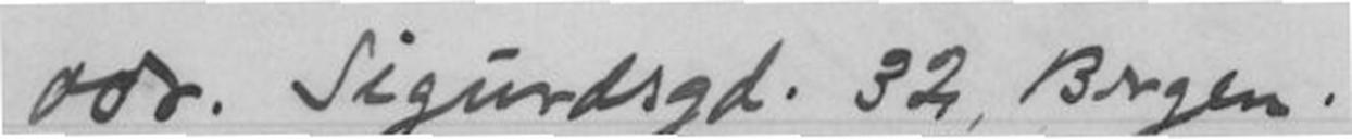adr. Sigurdgd. 32. Bergen.
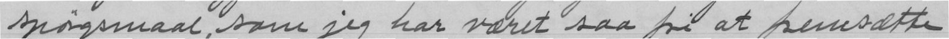spørgsmaal, som jeg har været saa fri at fremsætte
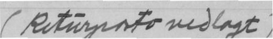(Returporto vedlagt)
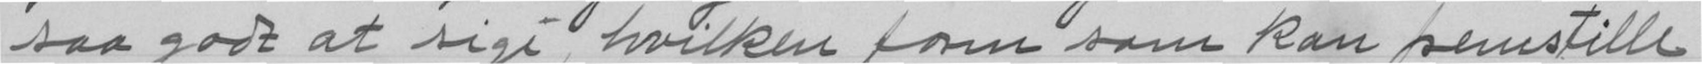saa godt at sige hvilken form som kan fremstille
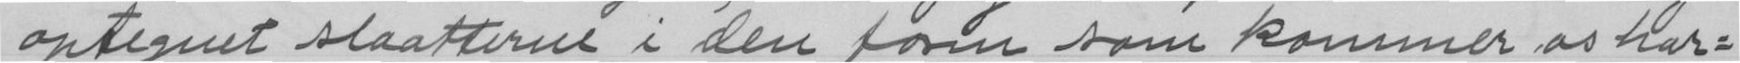optegnet slaatterne i den form som kommer os har-
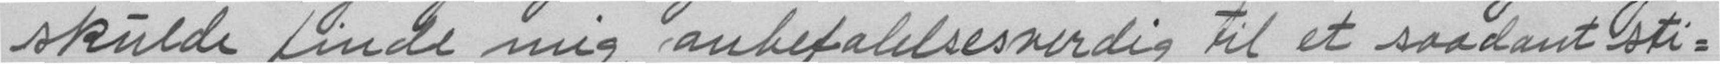skulde finde mig anbefalelsesverdig til et saadant sti-
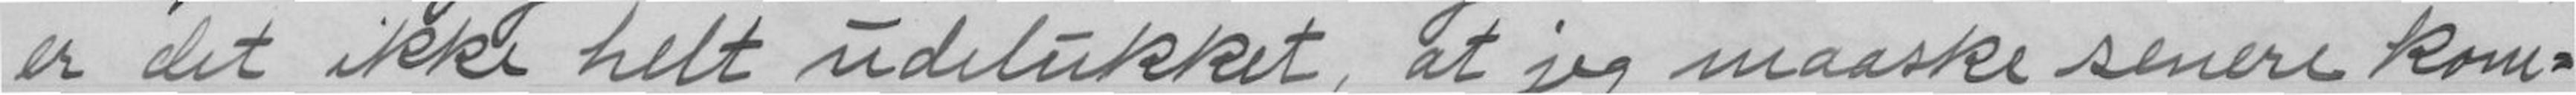er det ikke helt udelukket, at jeg maaske senere kom-
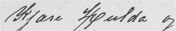Kjære Hulda og
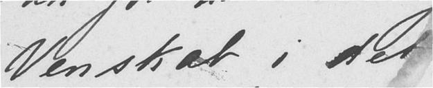Venskab i det
